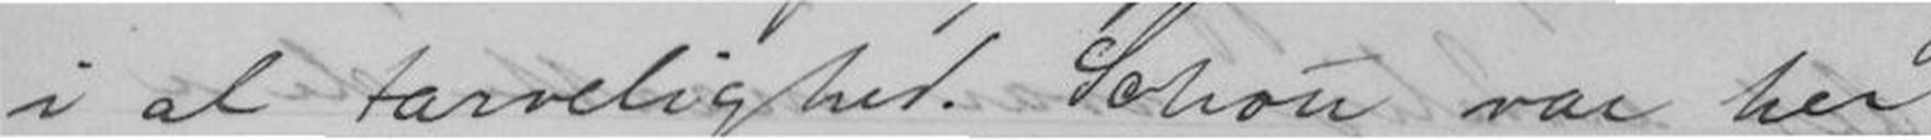i al tarvelighed. Schou var her
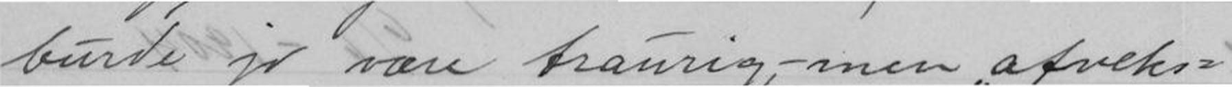burde jo være traurig, - men "afveks-
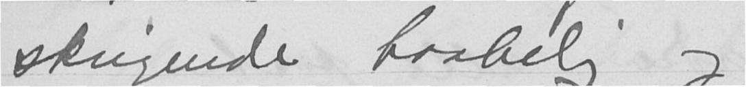skrigende taabelig og
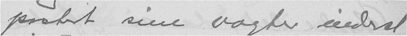postert sine vagter inderst
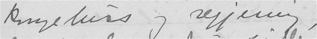kongehus og regjering,
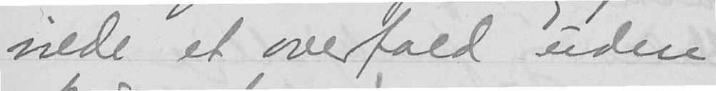vilde et overfald uden
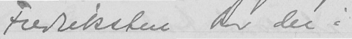Fredriksten har der i
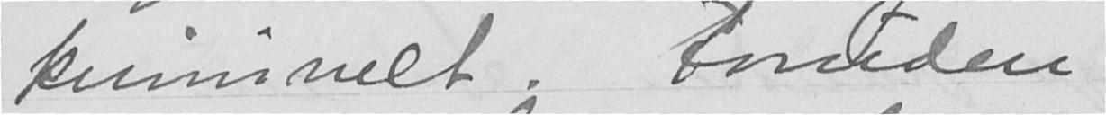kriminelt. Foruden
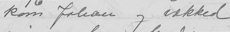kom Johan og vækked
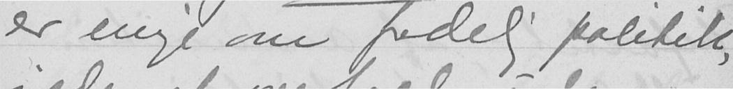er enige om fredelig politik,
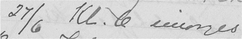27/6 Kl. 6 imorges
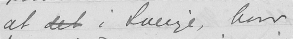at det i Sverige, hvor
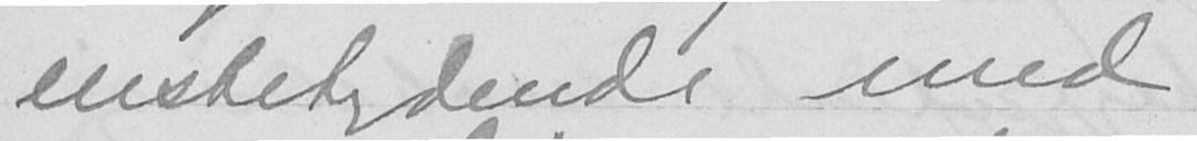ensbetydende med
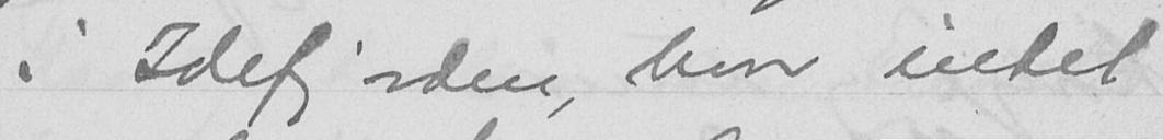i Idefjorden, hvor intet
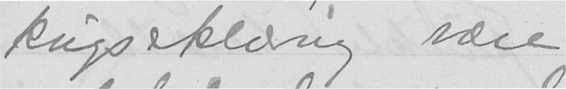krigserklæring være
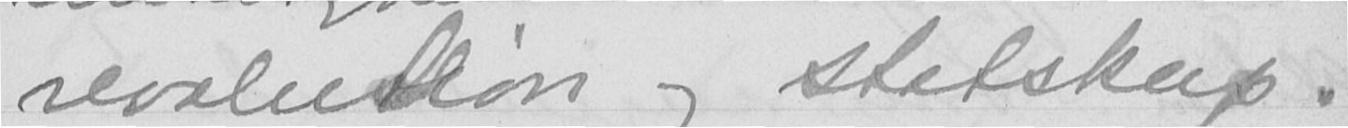revolution og statskup.
